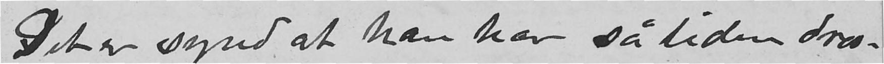Det er synd at han har så liden dres-
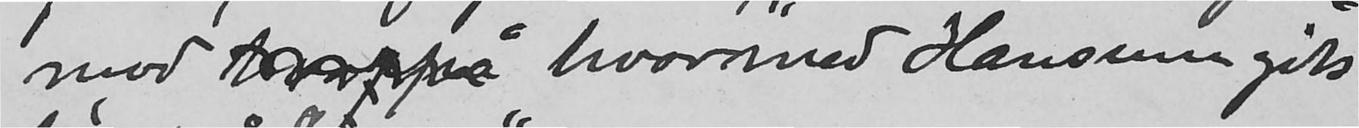mod derpå hvormed Hamsun gik
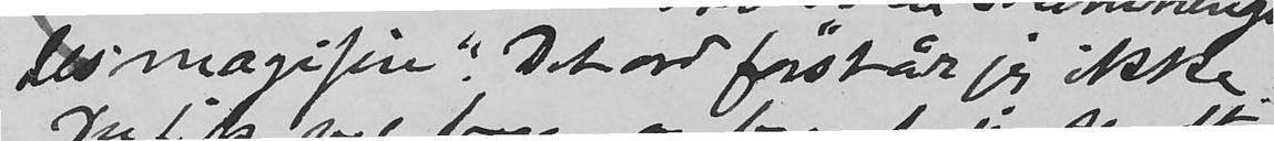des magisin". Det ord forstår jeg ikke
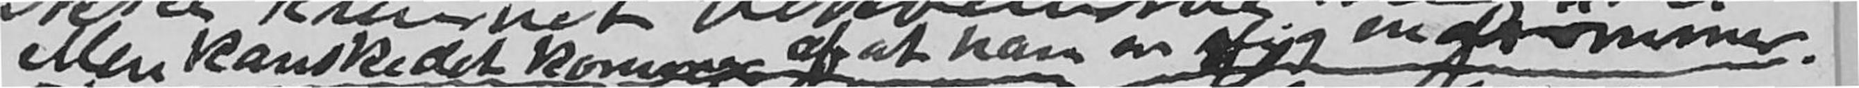eller kanske det kommer af at han er slig en drømmer.
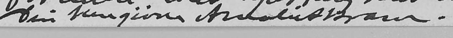Din hengivne Amalie Skram.
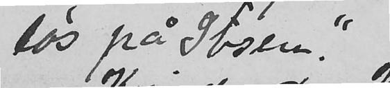løs på Ibsen."
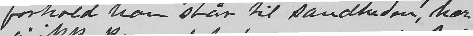forhold han står til sandheden, har
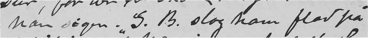han siger. G. B. slog ham flad på
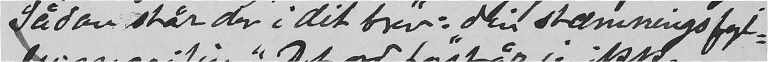Sådan står der i dit brev: "din stæmningsfyl-
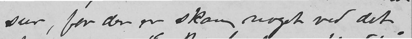sur, for der er skam noget ved det
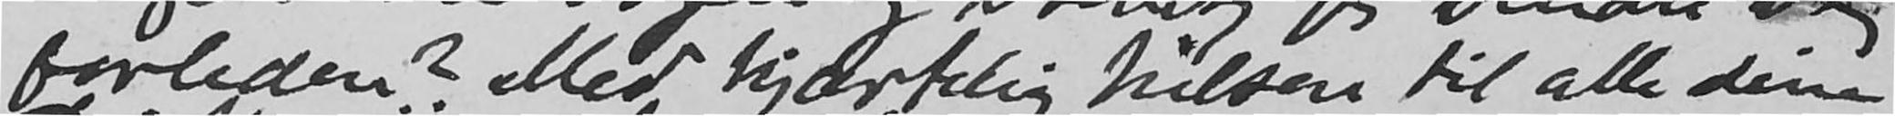forleden? Med hjærtelig hilsen til alle dine
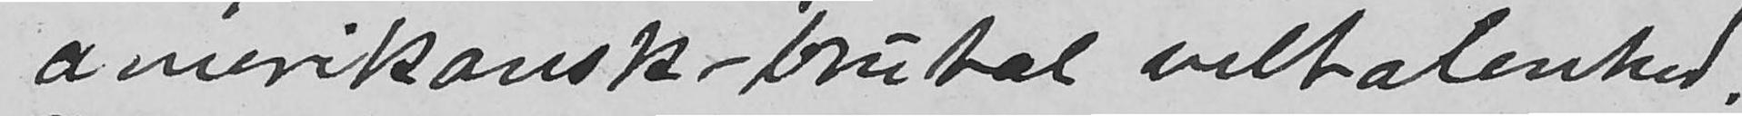amerikansk-brutal veltalenhed.
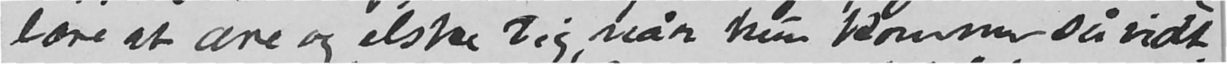lære at ære og elske Dig, når hun kommer så vidt
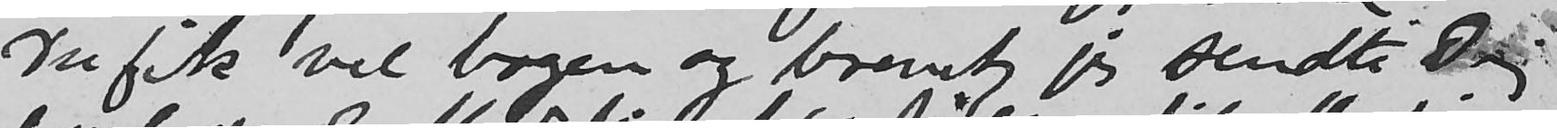Du fik vel bogen og brevet, jeg sendte Dig
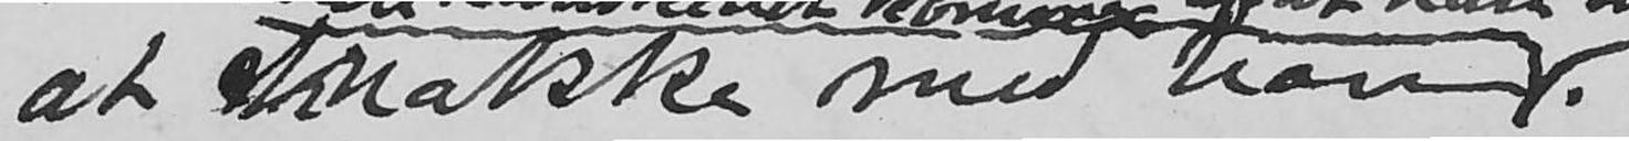at snakke med han.
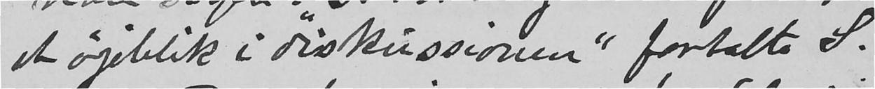et øjeblik i "diskussionen" fortalte S.
